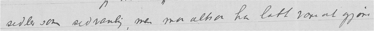sedler som sedvanlig, men maa altsaa ha latt være at gjøre
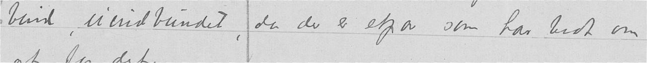bind, uindbundet, da der er etpar som har bedt om
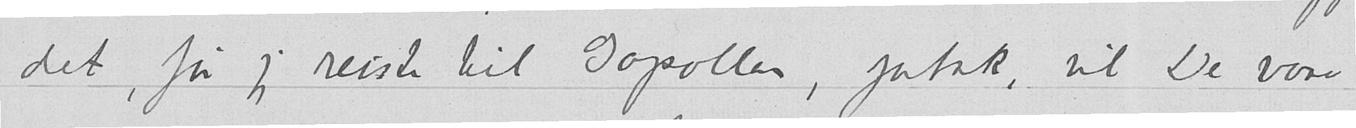det, før jeg reiste til Gopollen, jatak, vil De være
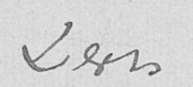Deres
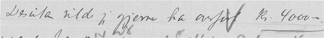Desuten vilde jeg gjerne ha overførf kr. 4000-
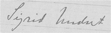Sigrid Undset
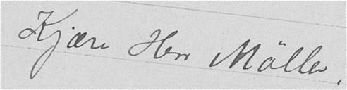Kjære Herr Møller,
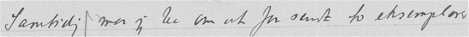Samtidig maa jeg be om at faa sendt to eksemplarer
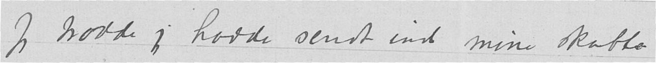Jeg trodde jeg hadde sendt ind mine skatte
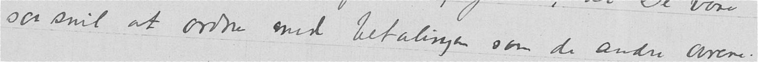saa snil at ordne med betalingen som de andre aarene.
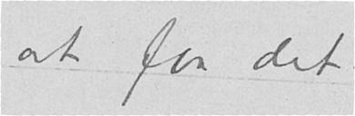at faa det
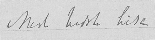Med bedste hilsen
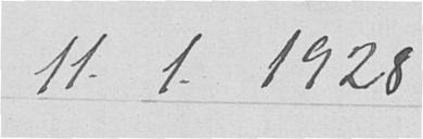11.1.1928
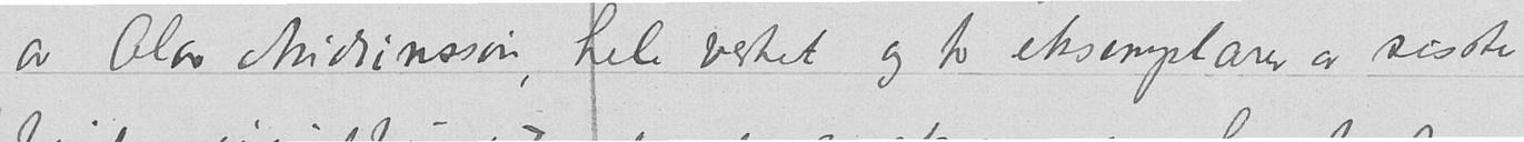av Olav Andunssøn, hele verket og to eksemplarer av sisste
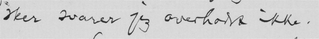sker svarer jeg overhodet ikke.
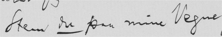Stem du paa mine Vegne
